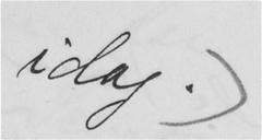idag.
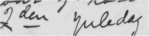2den Juledag
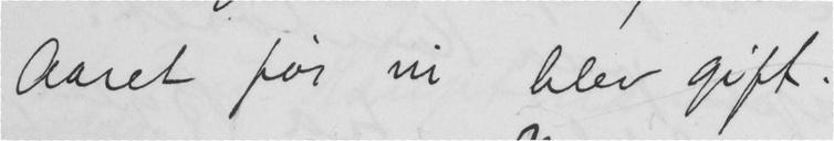Aaret før vi blev gift.
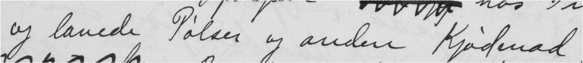og lavede Pølser og anden Kjødmad
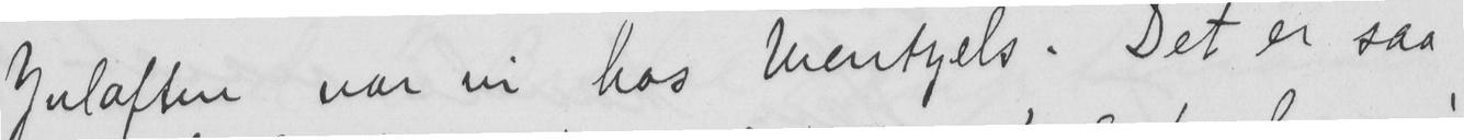Julaften var vi hos Wentzels. Det er saa
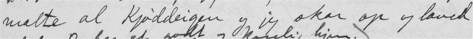malte al Kjøddeigen og jeg skar op og laved
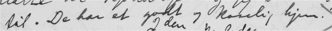til. De har et godt og koselig hjem.
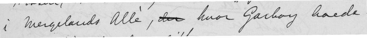i Wergelands Allè,  hvor Garborg boede
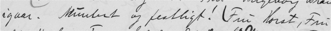igaar. Muntert og festligt! Fru Horst, Fru
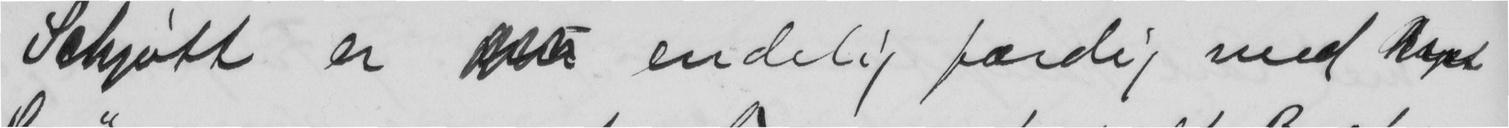Schjøtt er  endelig færdig med kapt
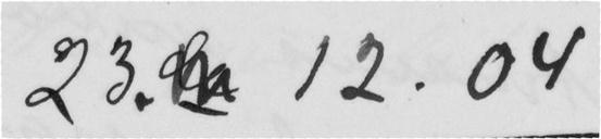23. 12.04
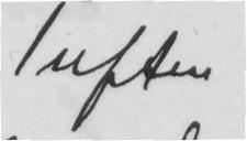Tuften
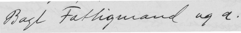Bagt Fattigmand og a.
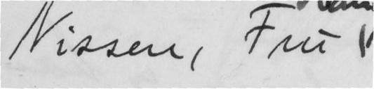Nissen, Fru
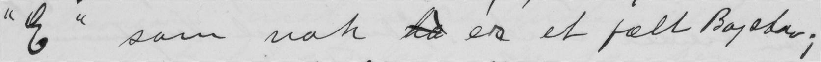"E" som nok  er et fælt Bogstav;
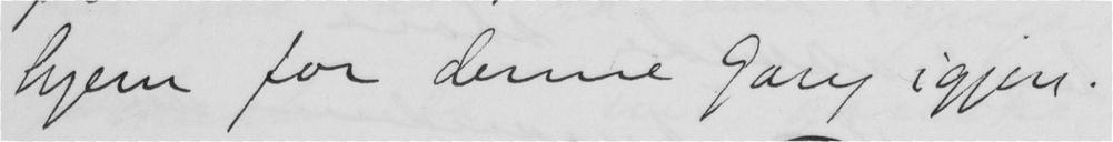hjem for denne Gang igjen.
